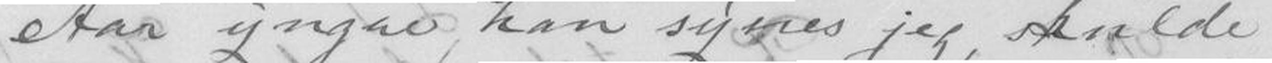Aar yngre, han synes jeg, skulde
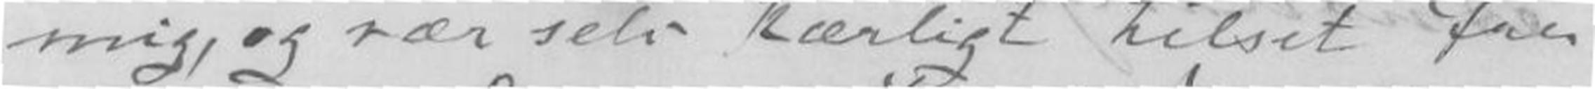mig, og vær selv kærligt hilset fra
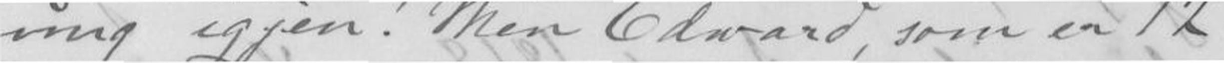ung igjen! Men Edward, som er 12
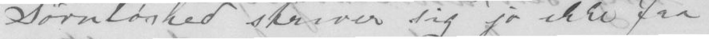Søvnløshed skriver sig jo ikke fra
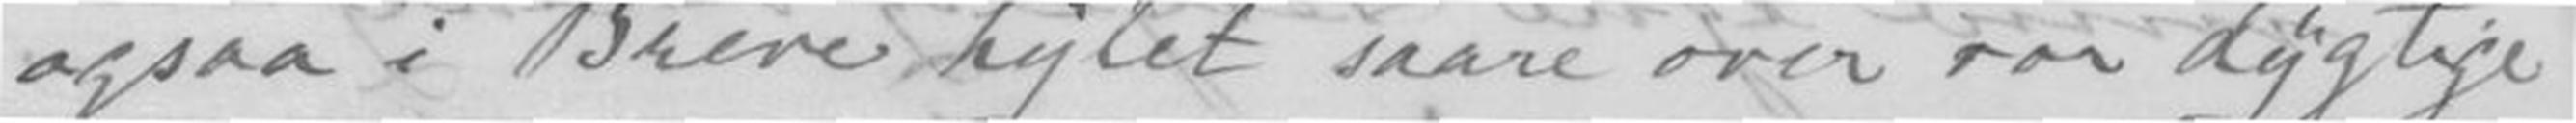ogsaa i Breve hylet saare over vor dygtige
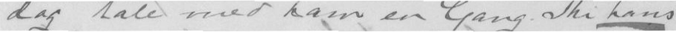dog tale med ham en Gang. Thi hans
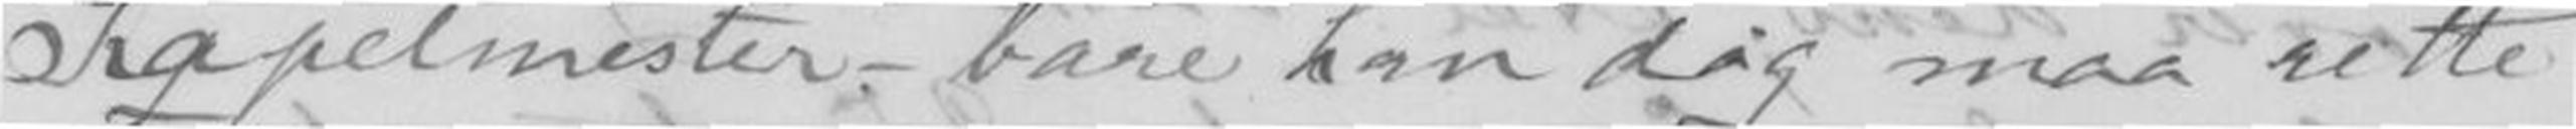Kapelmester - bare han dog maa rette
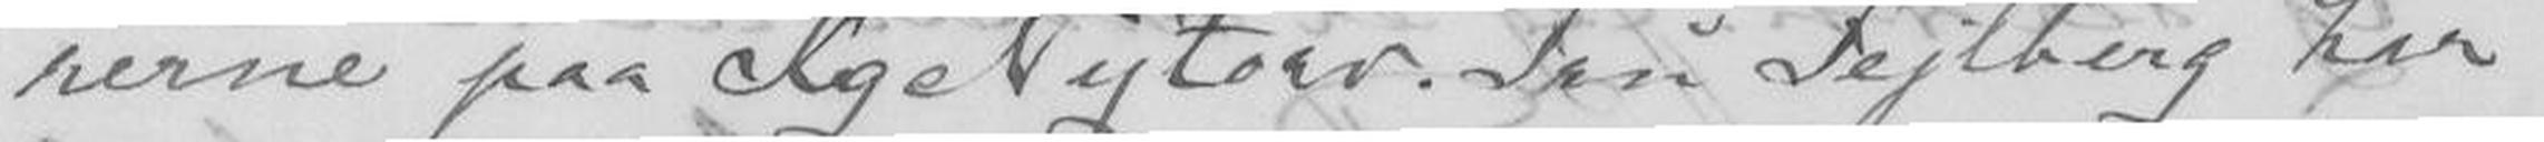rerne paa Kg Nytorv. Fru Fejlberg har
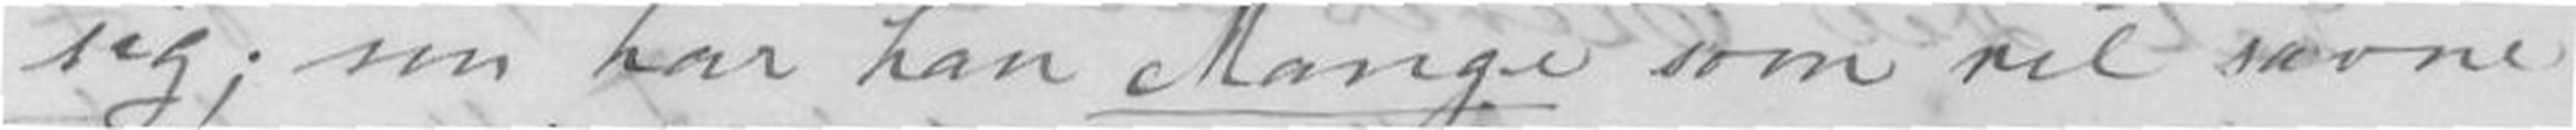sig; nu har han Mange som vil savne
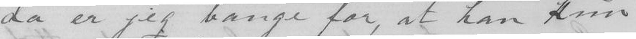da er jeg bange for, at han kun
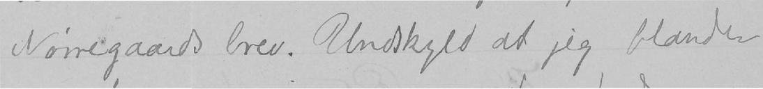Nørregaards brev. Undskyld at jeg blander
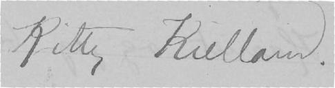Kitty Kielland.
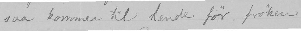saa kommer til hende før frøken
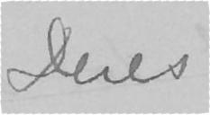Deres
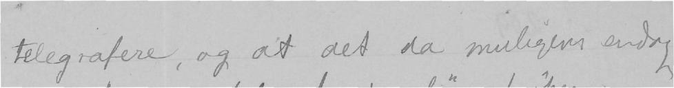telegrafere, og at det da muligens endog
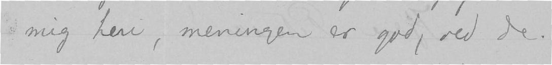mig heri, meningen er god, ved de.
